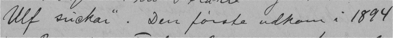Ulf suckar". Den første udkom i 1894
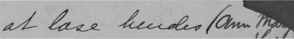at læse hendes (Ann Marg
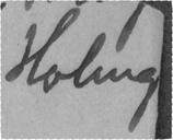Holmg
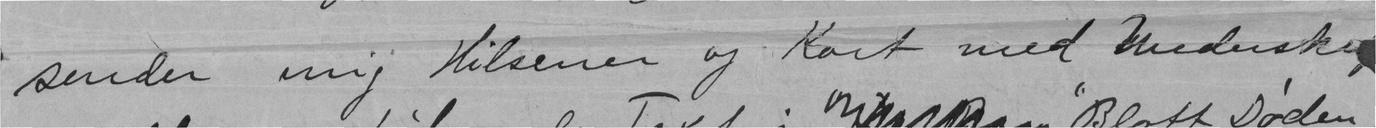sender mig Hilsener og kort med Underskrift
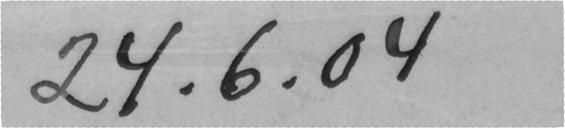24.6.04
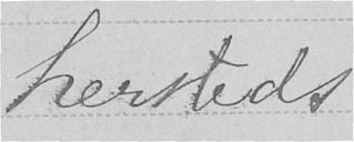hersteds
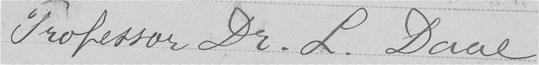Professor Dr. L. Daae
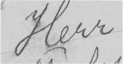Herr
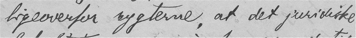ligeoverfor rygterne, at det juridiske
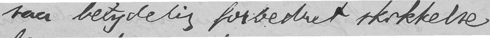saa betydelig forbedret skikkelse
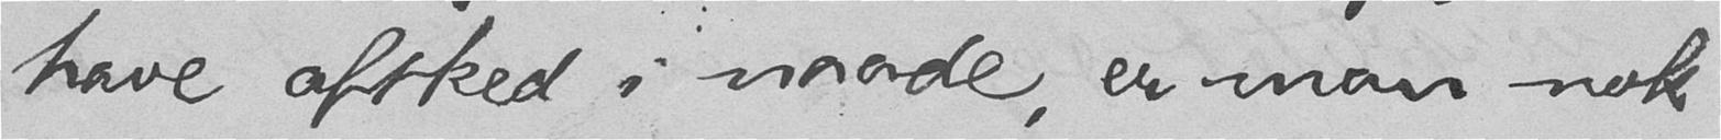have afsked i naade, er man nok
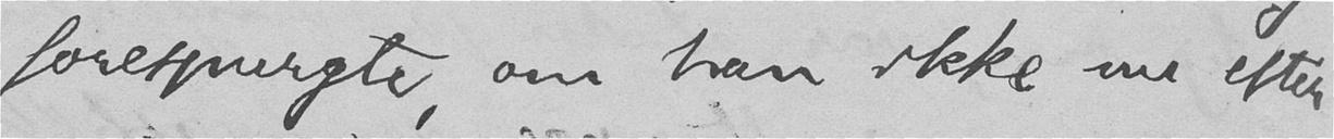forespurgte, om han ikke ne efter
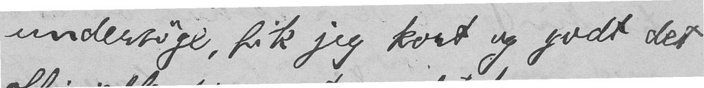undersøge, fik jeg kort og godt det
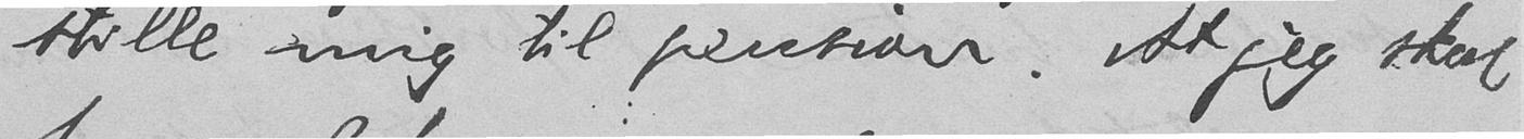stille mig til pension. At jeg skal
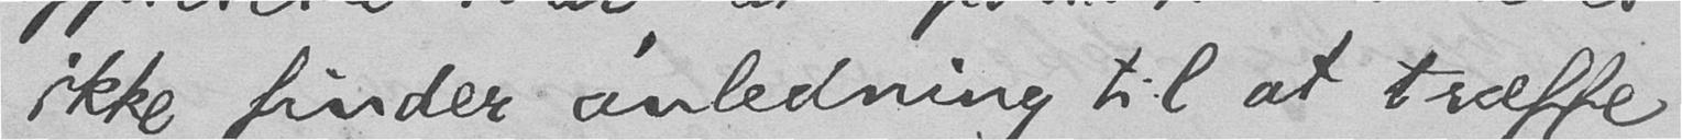ikke finder anledning til at træffe
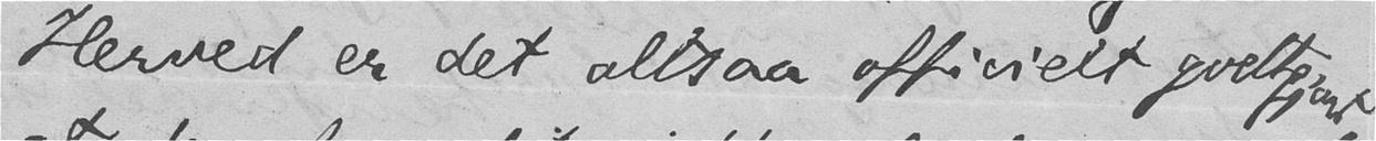Herved er det altsaa officielt godtgjort,
